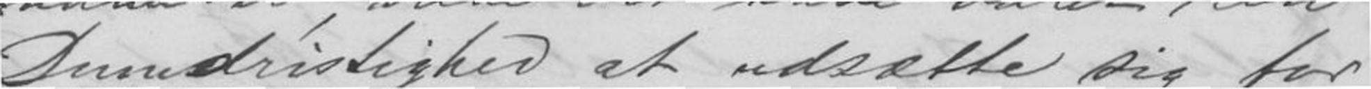Dumdristighed at udsætte sig for
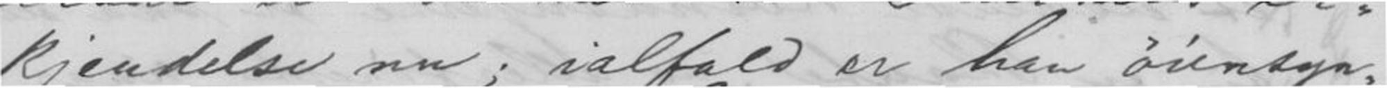kjendelse nu; ialfald er han øiensyn-
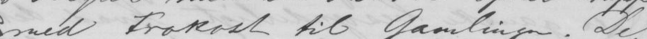med Frokost til Gamlingen. De
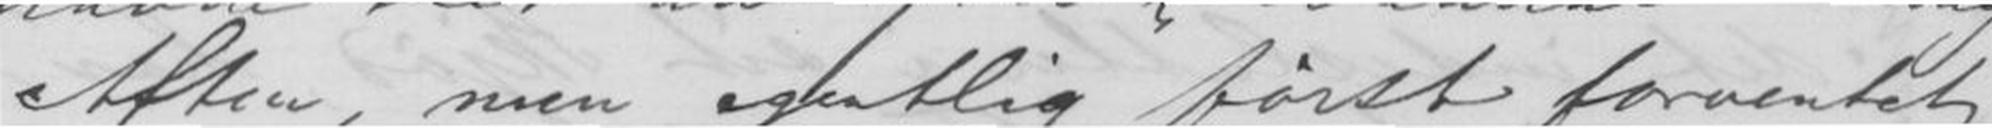Aften, men egentlig først forventet
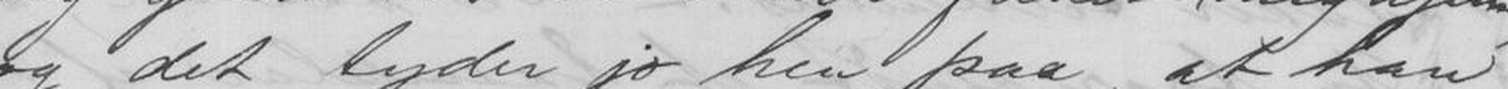og det tyder jo hen paa, at han
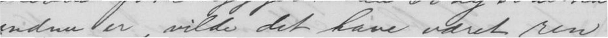endnu er, vilde det have været ren
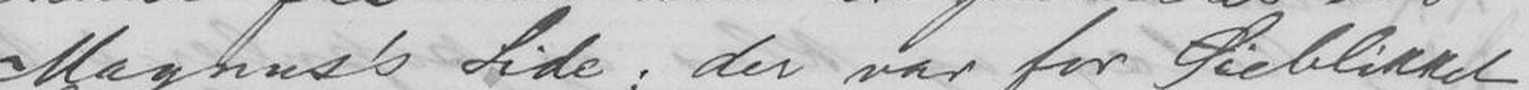Magnus's Side; der var for Øieblikket
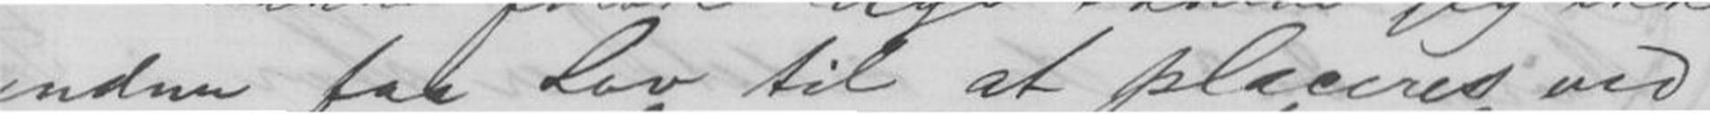endnu faa Lov til at placeres ved
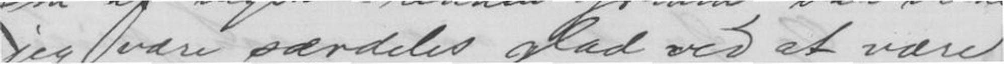jeg være særdeles glad ved at være
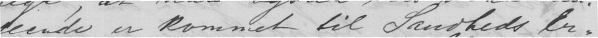seende er kommet til Sandheds Er-
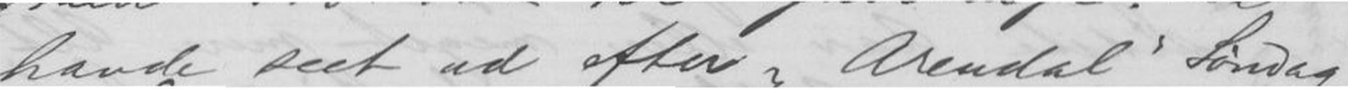havde seet ud efter Arendal Søndag
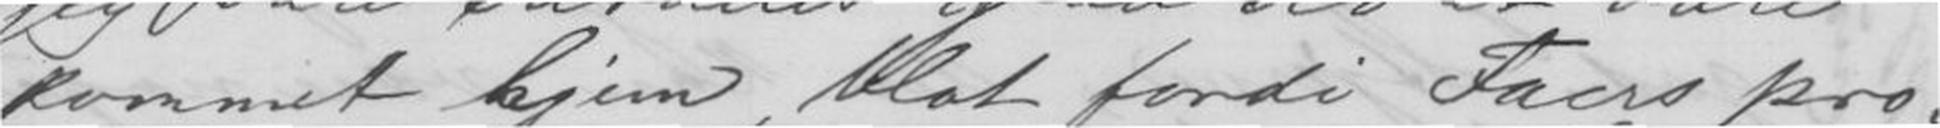kommet hjem, blot fordi Faers pro-
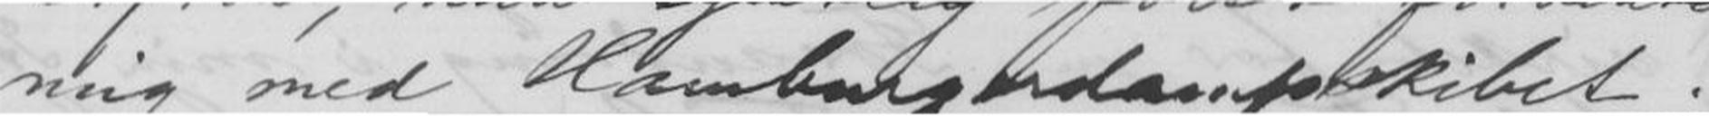mig med Hamburgerdamskibet.
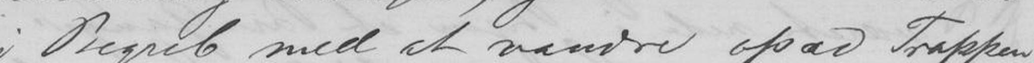i Begreb med at vandre opad Trappen
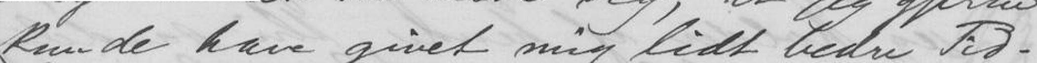kunde have givet mig lidt bedre Tid.
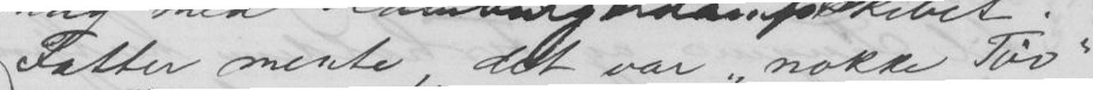Fatter mente, det var nokke Tøv,
